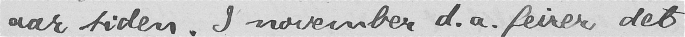aar siden. I november d.a. feirer det
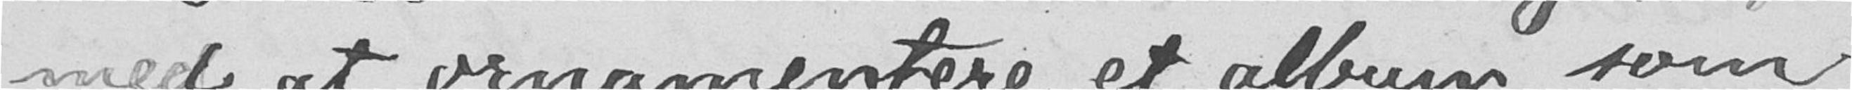med at ornamentere et album, som
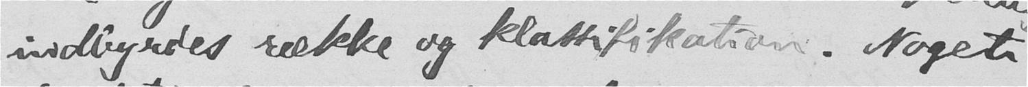indbyrdes række og klassifikation. Noget
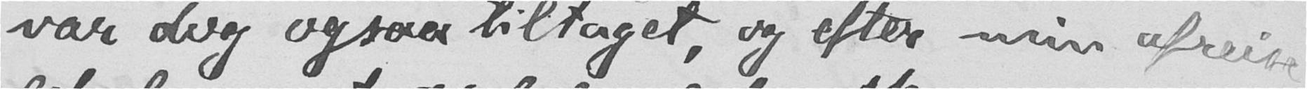var dog ogsaa tiltaget, og efter min afreise
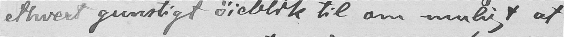ethvert gunstigt øieblik til om muligt at
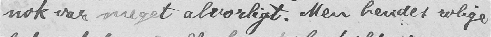nok var meget alvorligt. Men hendes rolige
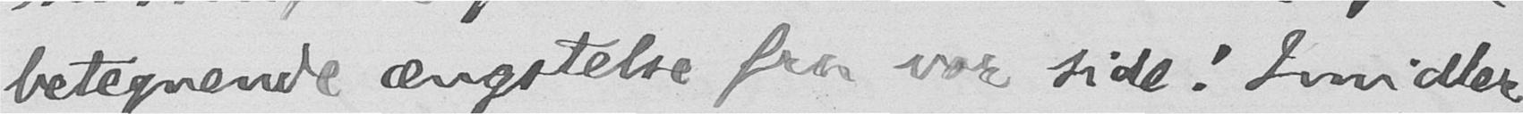betegnende ængstelse fra vor side! Imidler-
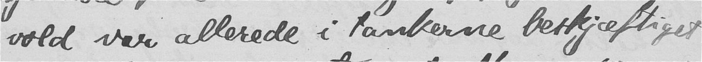vold var allerede i tankerne beskjæftiget
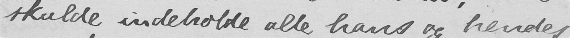skulde indeholde alle hans og hendes
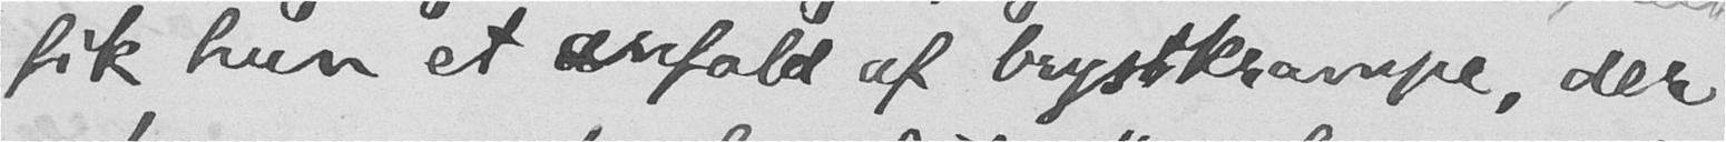fik hun et anfald af brystkrampe, der
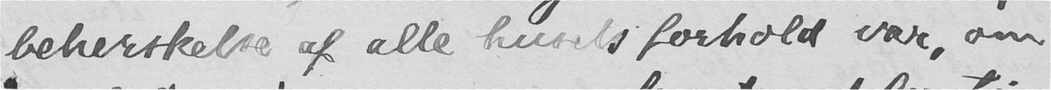beherskelse af alle husets forhold var, om
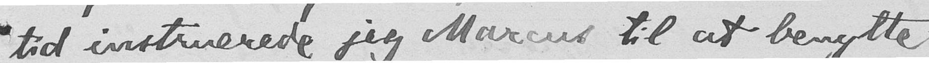tid instruerede jeg Marcus til at benytte
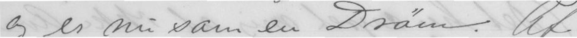og er nu som en Drøm. Af
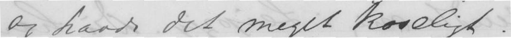og havde det meget koseligt.
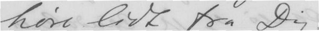høre lidt fra Dig.
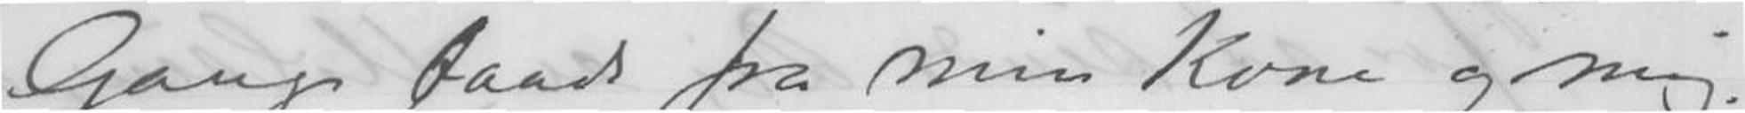Gange baade fra min Kone og mig.
